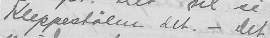Kleppestølen det. - Det
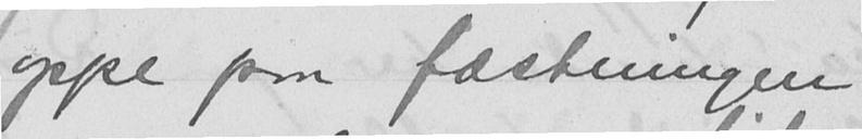oppe paa fæstningen
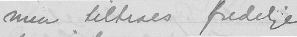men tiltroes fredelige
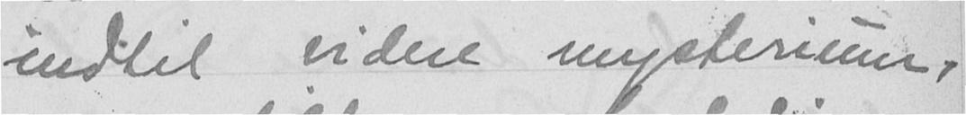indtil videre mysterium,
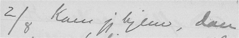2/8 Kom jeg hjem, Don
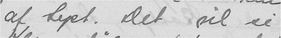af Sept. Det vil si
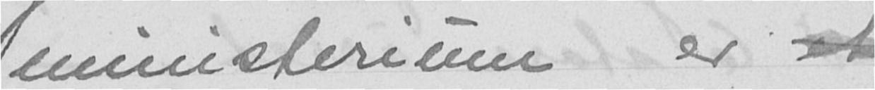ministerium er et
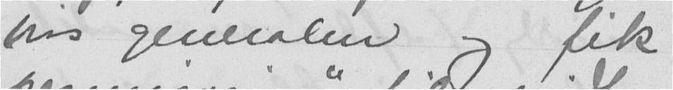hos generalen og fik
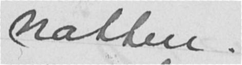natten.
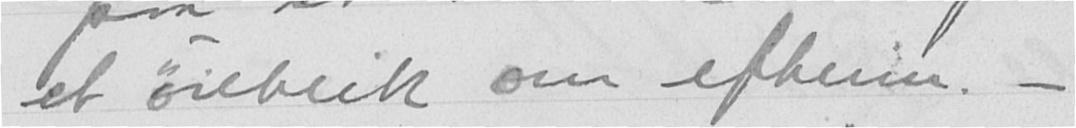et øieblik om efterm. -
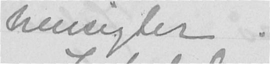hensigter.
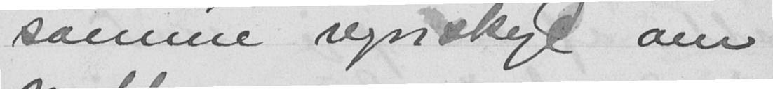somme regnskyl om
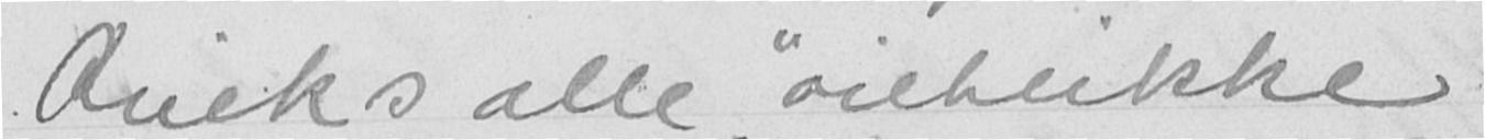Riecks alle øieblikke.
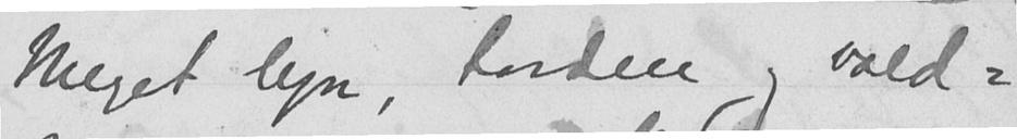Meget lyn, torden og vold-
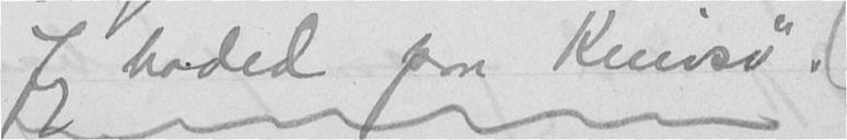Jeg baded paa Knivsø.
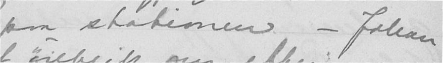paa stationen - Johan
